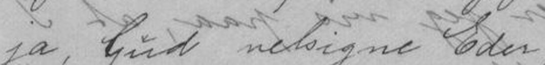ja, Gud velsigne Eder,
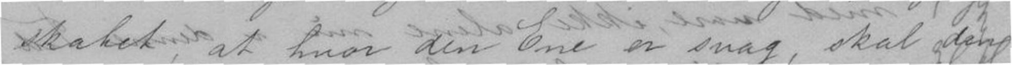skabet, at hvor den Ene er svag, skal den
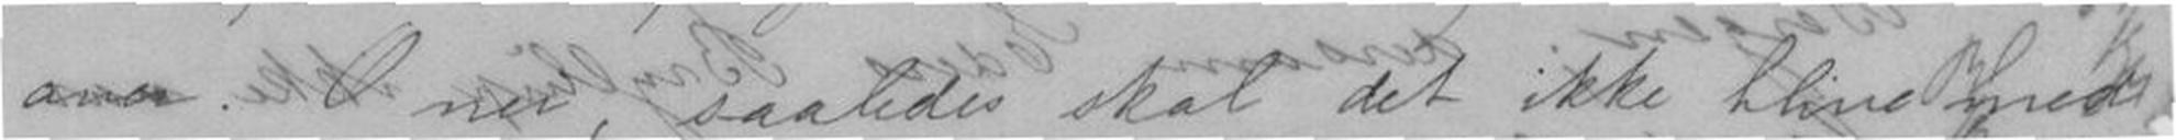over. O nu, saaledes skal det ikke blive med
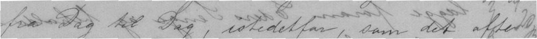fra Dag til Dag, istedetfor, som det oftes
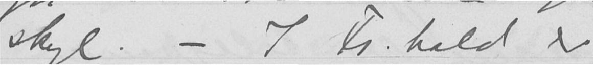skyl. - I Fr. hald er
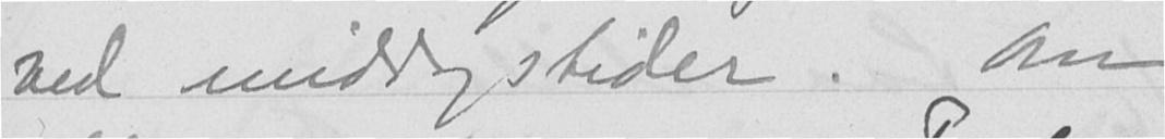ved middagstider. Om
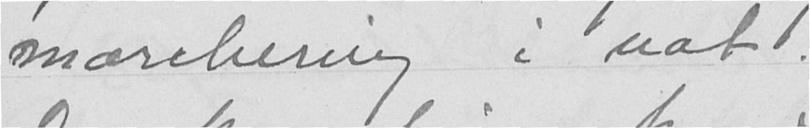marchering i nat.
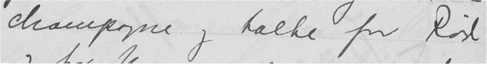champagne og talte for Rød
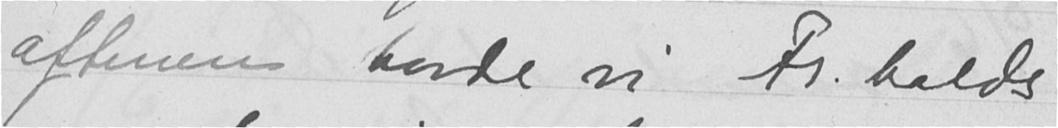aftenen havde vi Fr. halds
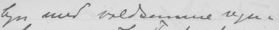lyn med voldsomme regn-
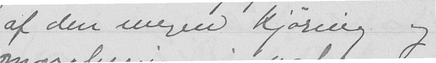af den megen kjøring og
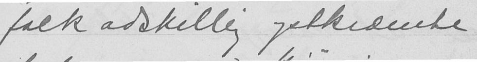folk adskillig opskræmte
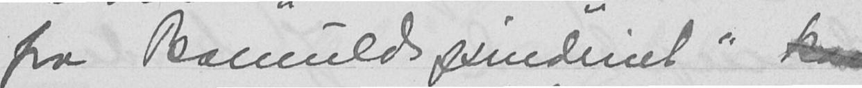fra Bomuldspinderiet" kan
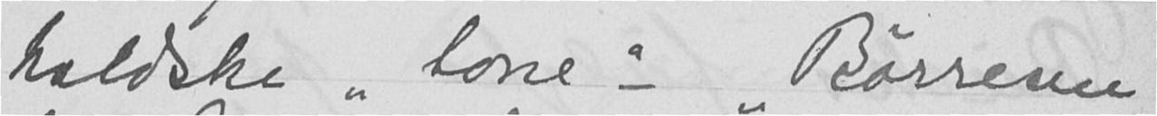haldske "tone" - "Børresen
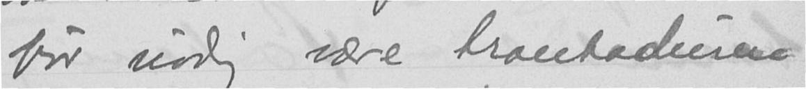bør nødig være troubaduren
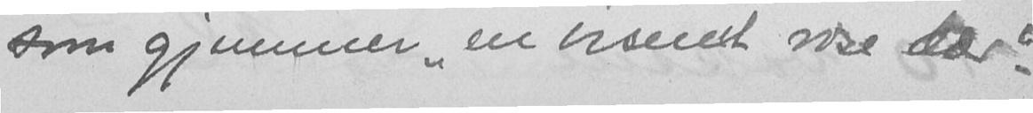som gjemmer "en visnet rose dær."
